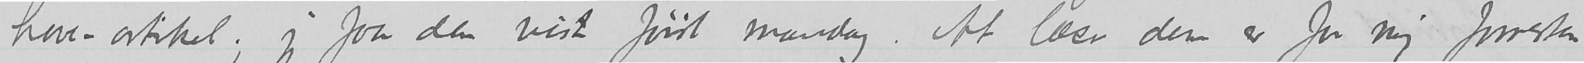have-artikel; jeg faar den vist først mandag. At læse den er for mig forresten
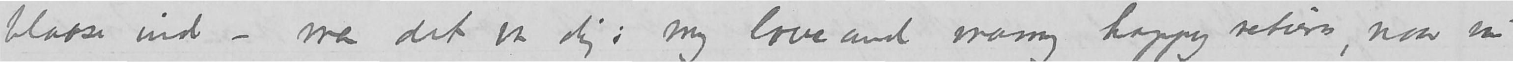blaase ind – men det varer dig: my love and many happy returns, naar nu
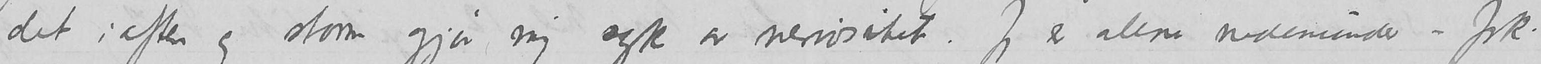det i aften og storm gjør mig syk av nervøsitet.  Jeg er alene nedenunder – frk.
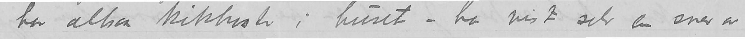Jeg har altsaa kikhoste i huset – har vist selv en snev av
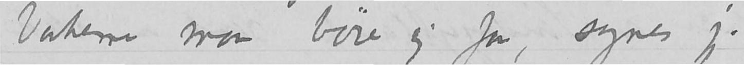Vorherre maa bøie sig for, synes jeg.
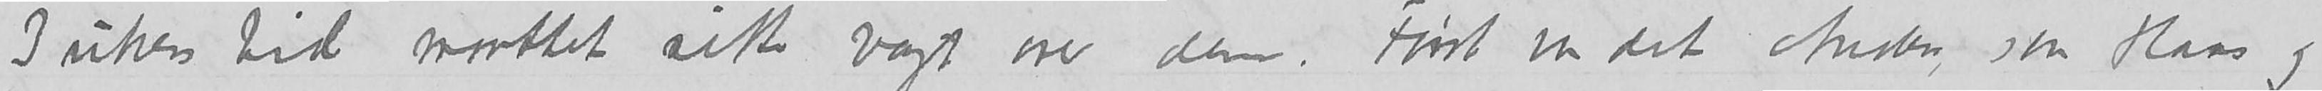tre ukers tid maattet sitte vagt over dem.  Først vaer det Anders, saa Hans og
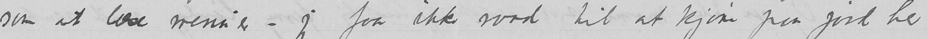som at læse menuer – jeg faar ikke raad til at kjøre paa jord her
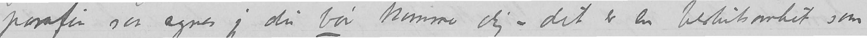parafin saa synes jeg du bør komme dig – det er en beslutsomhet som
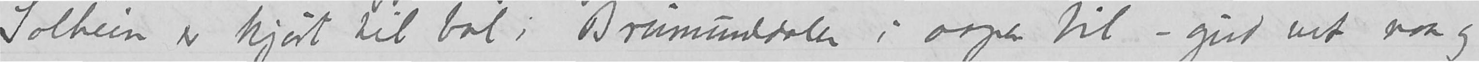Solheim er kjørt til bal i Brumunddalen i aapen bil – gud vet naar og
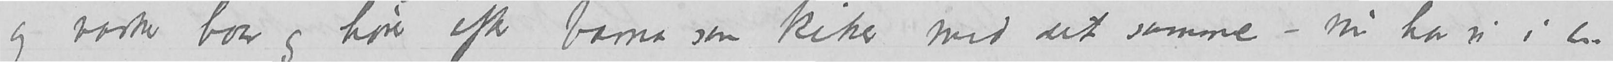og vasker tøi og hører efter barna som kiker med det samme – nu har vi i en
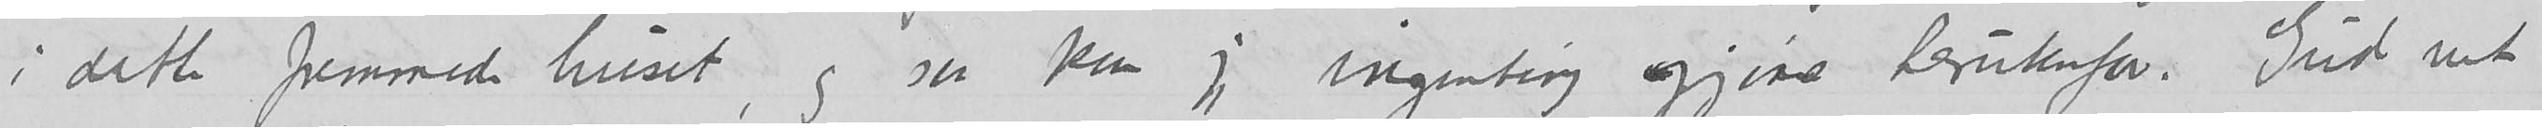i dette fremmede huset, og saa kan jeg ingenting gjøre herutenfor. Gud vet
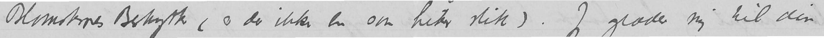Blomsternes Beskytter (er der ikke en som heter slik). Jeg glæder mig til din
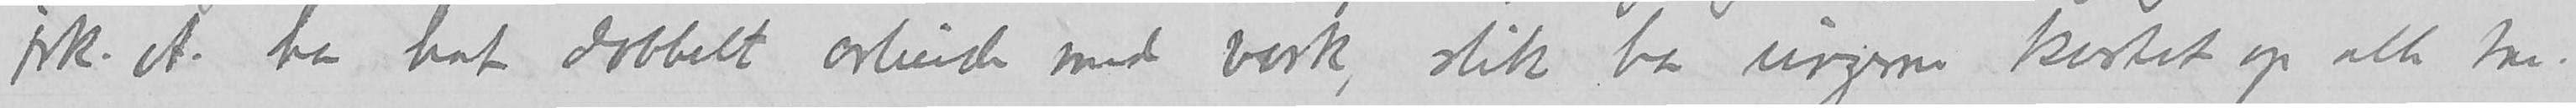Frk. A. har hat dobbelt arbeide med vask, slik har ungerne kastet op alle tre.
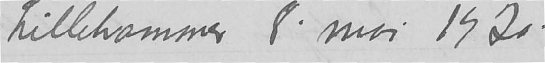Lillehammer 8. mai.1920
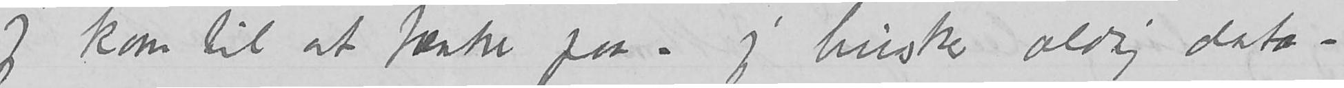jJeg kom til at tænke paa – jeg husker aldrig dato -
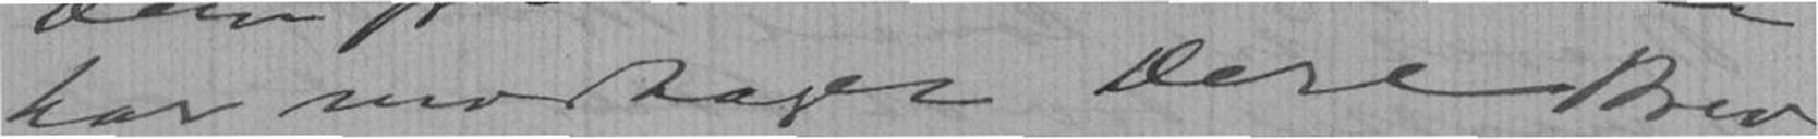har modtaget Deres Brev
